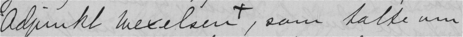Adjunkt Wexelsen, som talte om
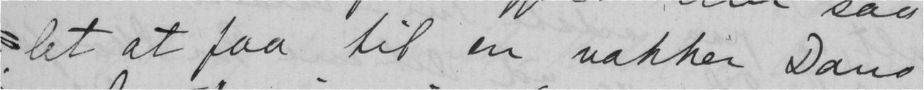let at faa til en vakker Dans
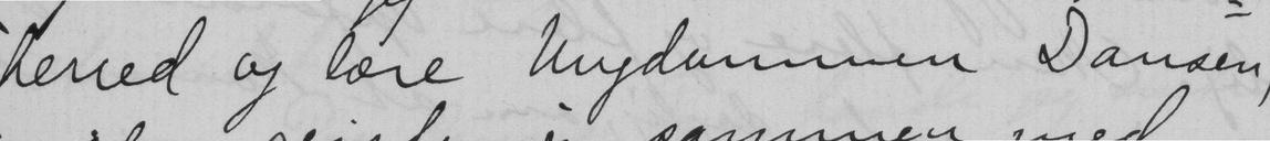herred og lære Ungdommen Dansen,
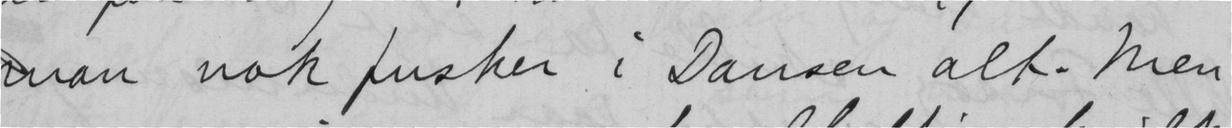man nok fusker i Dansen alt. Men
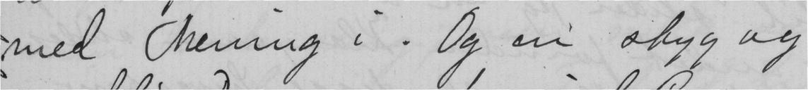med Mening i. Og en styg og
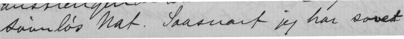søvnløs Nat. Saasnart jeg har sovet
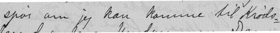spør om jeg kan komme til Krøds-
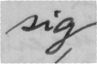sig
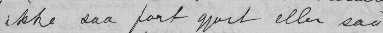ikke saa fort gjort eller saa
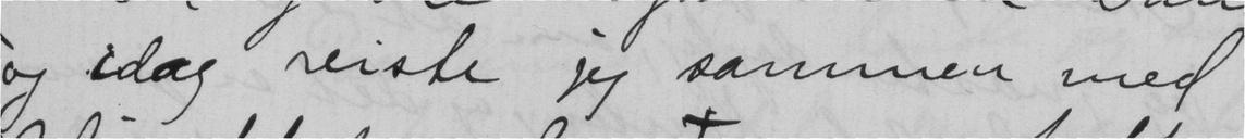og idag reiste jeg sammen med
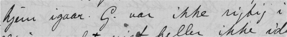hjem igaar. G. var ikke rigtig i
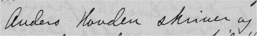Anders Hovden skriver og
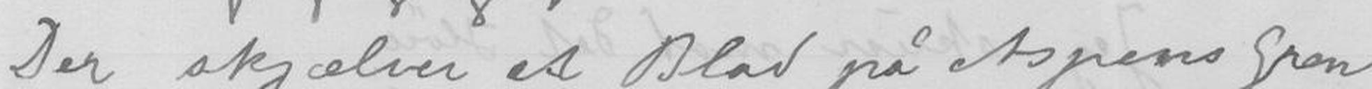Der skjælver et Blad på Aspens Gren
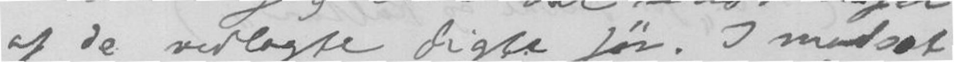af de vedlagte digte før. I modsat
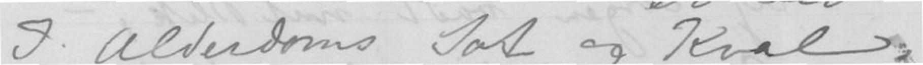I Alderdoms Sot og Kval.
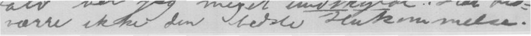værre ikke den bedste Hukommelse.
